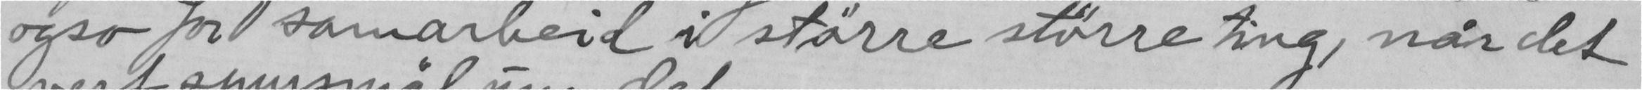ogso for samarbeid i større større ting, når det
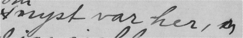nyst var her, og
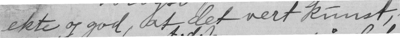ekte og god, at det vert kunst,
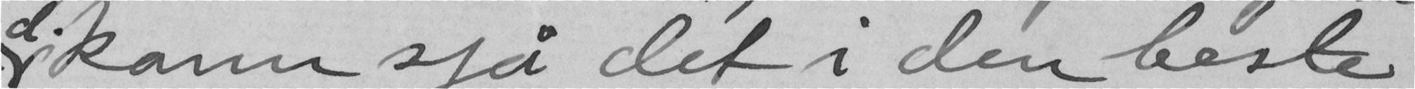kann sjå det i den beste
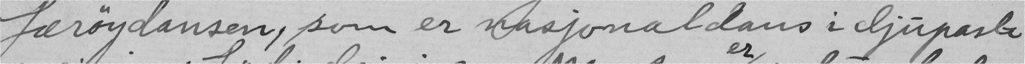færøydansen, som er nasjonaldans i djupaste
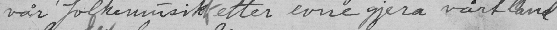vår folkemusikk etter evne gjera vårt land
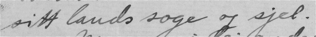sitt lands soge og sjel.
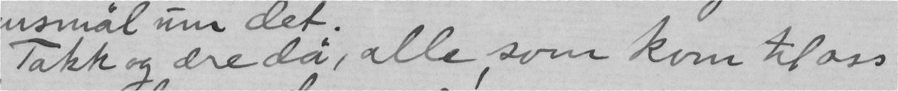Takk og ære då, alle, som kom til oss
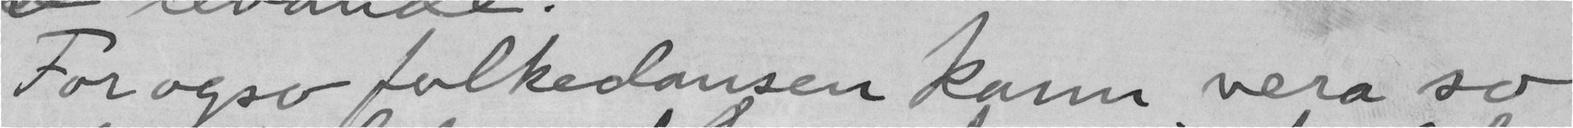For ogso folkedansen kann vera so
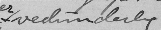vedunderleg
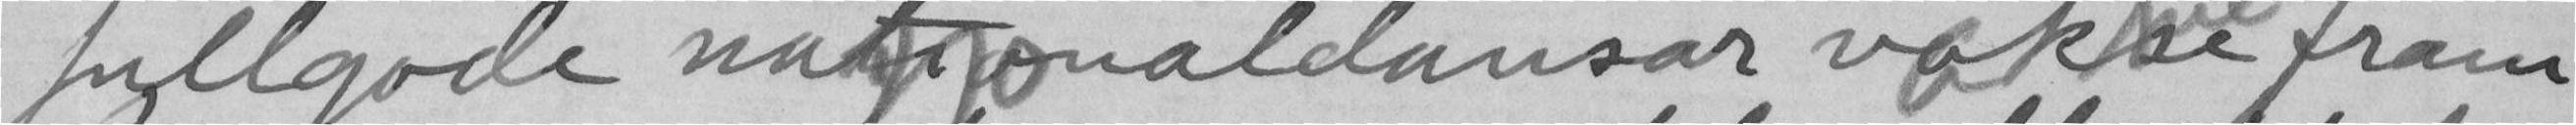fullgode nationaldansar vakse fram
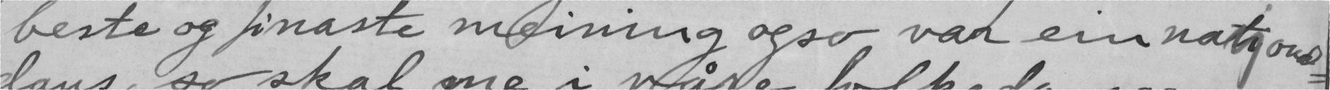beste og finaste meining ogso var ein national-
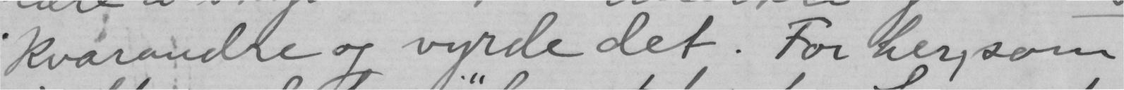kvarandre og vyrde det. For her, som
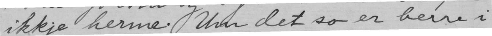ikkje herme. Um det so er berre i
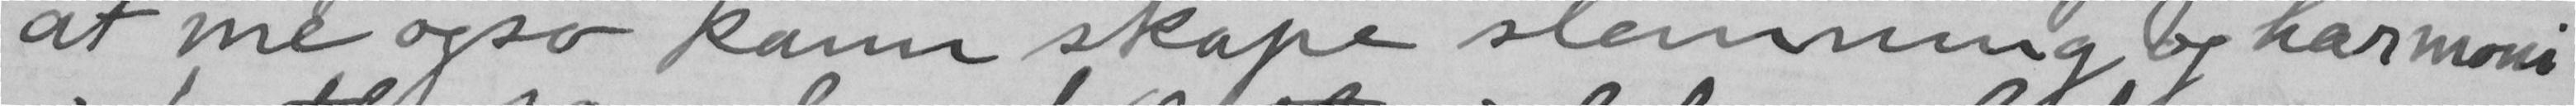at me ogso kann skape stemning og harmoni
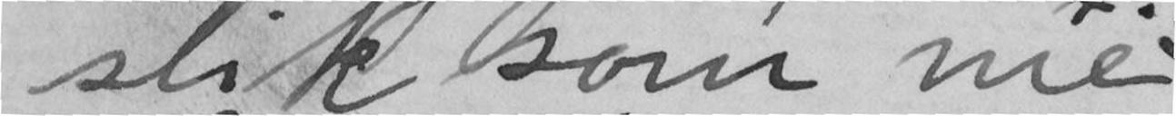slik som me
